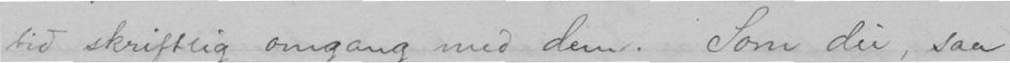tid skriftlig omgang med dem. Som du, saa
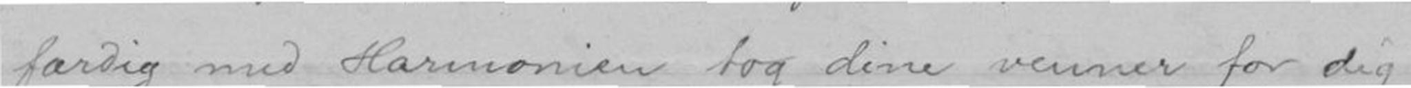færdig med harmonien tog dine venner for dig
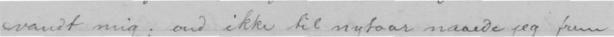vandt mig; end ikke til nytaar naede jeg frem
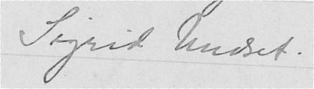Sigrid Undset.
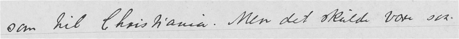som til Christiania. Men det skulde være saa
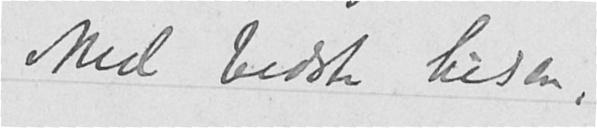Med bedste hilsen,
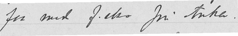faa med f.eks. fru Anker.
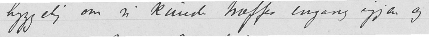hyggelig om vi kunde træffes engang igjen og
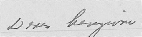Deres hengivne
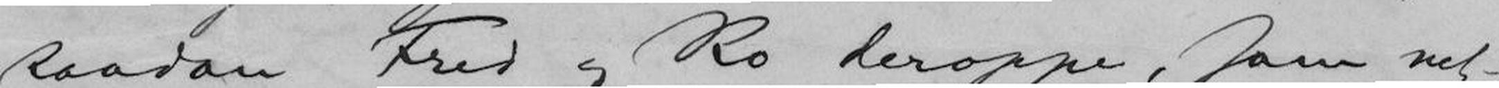saadan Fred og Ro deroppe, som net-
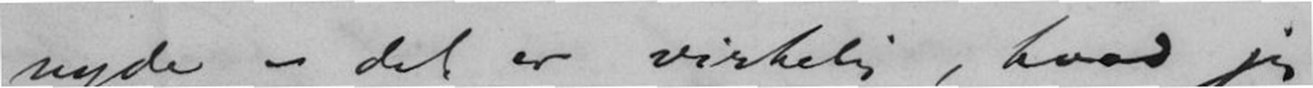nyde - det er virkelig, hvad jeg
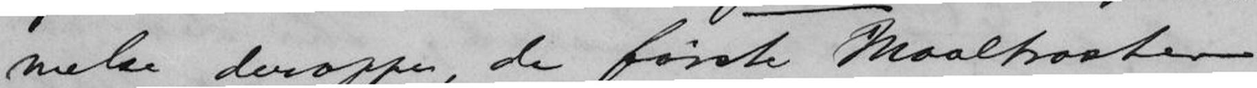melse deroppe, de første Maaltroster
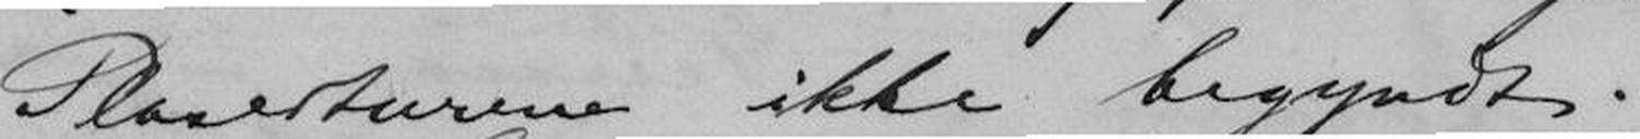Plaserturene ikke begyndt.
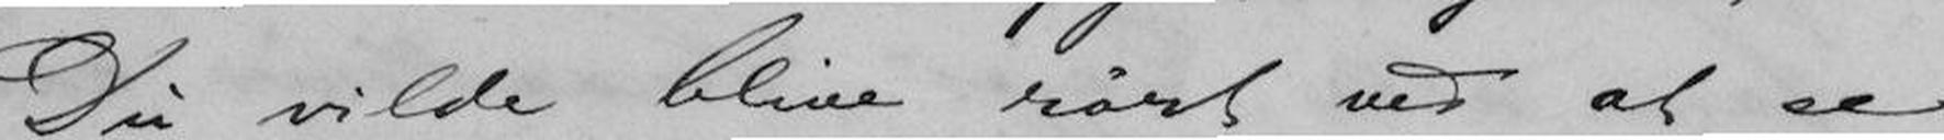Du vilde blive rørt ved at se
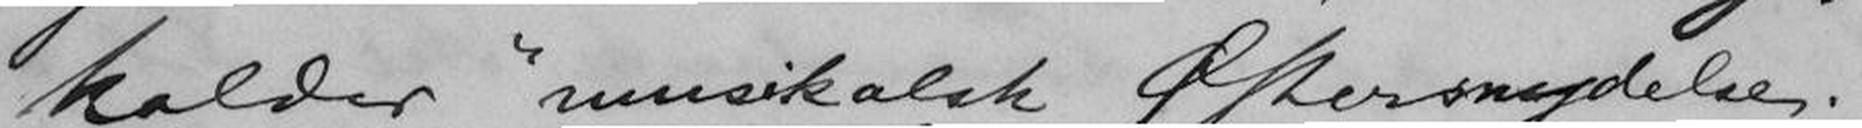kalder "musikalsk Østersnydelse."
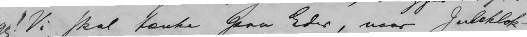ge! Vi skal tænke paa Eder, naar Juleklok-
